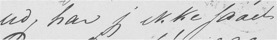ud, har jeg ikke faaet
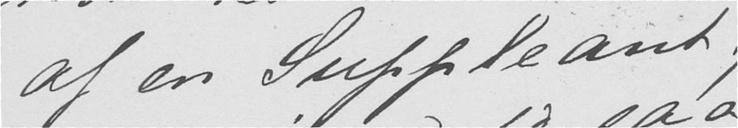af en Suppleant;
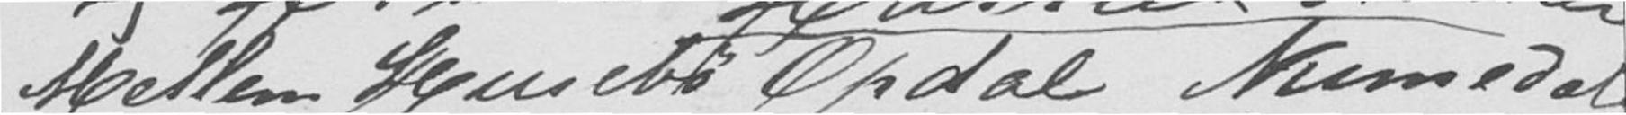Mellem Husebø Opdal Numedal
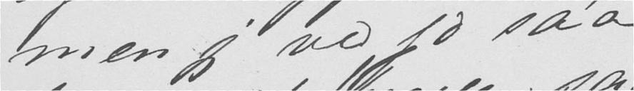men jeg ved jo saa
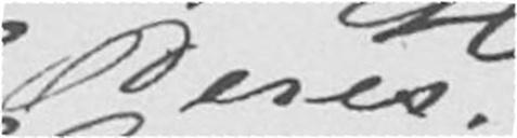Deres
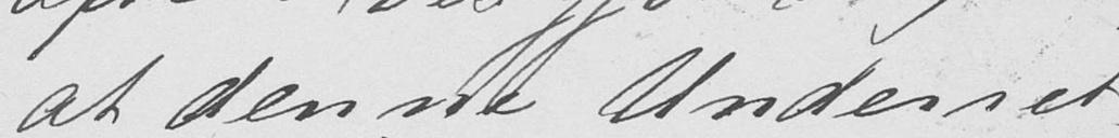at denne Underret-
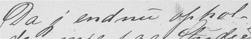Da jeg endnu ophol-
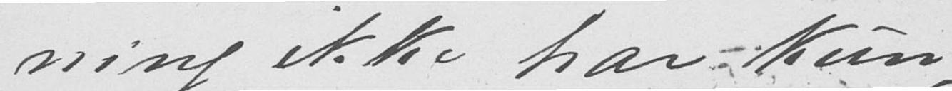ning ikke har kun-
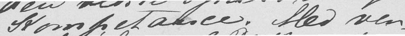Kompetance. Med ven-
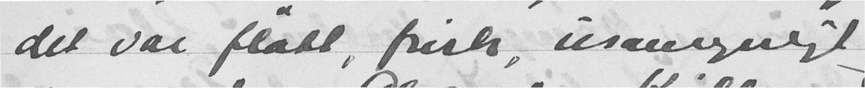det var flott, frisk, usansynligt
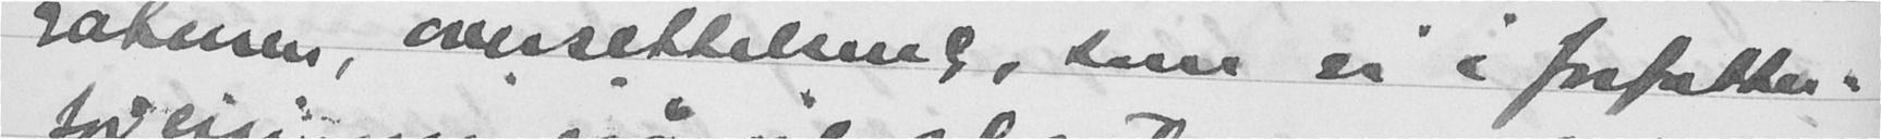raturen, oversettelsene, som vi i forfatter-
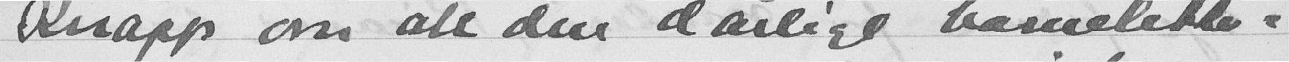Knapp om all den dårlige barnelitte-
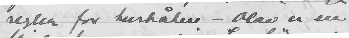regler for turbåten - Olav er en
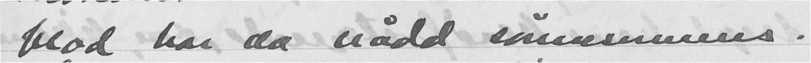blod har da nådd sønnesønnens.
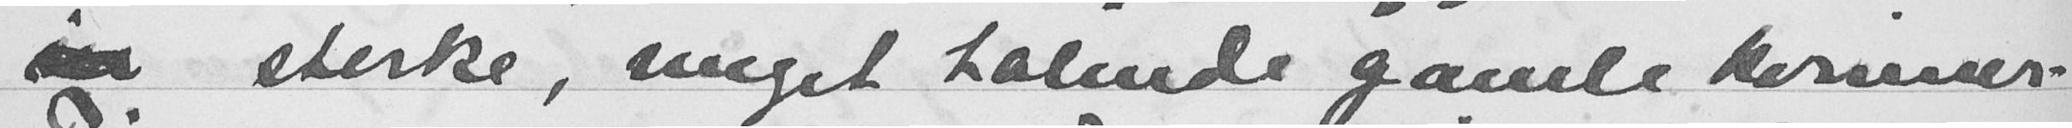sterke, meget talende gamle kvinner.
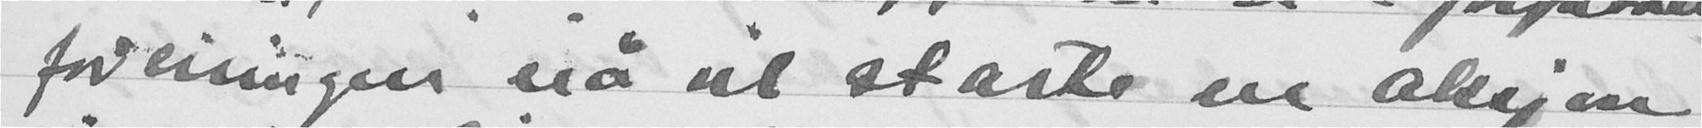foreningen nå vil starte en aksjen
